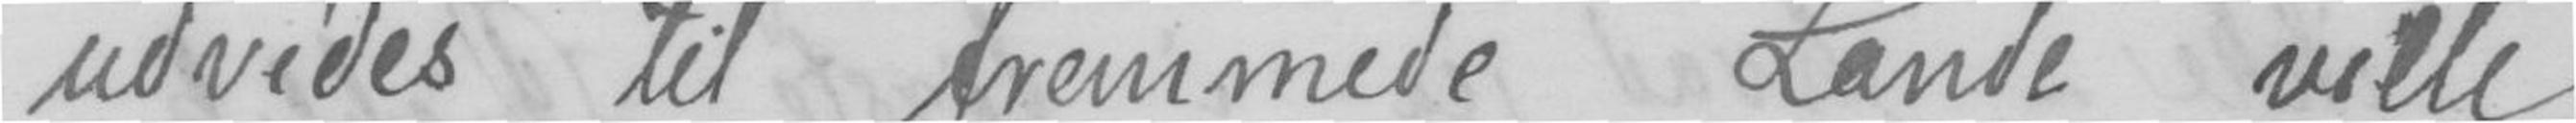udvides til fremmede Lande ville
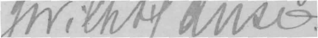Wilhelm, Hansen
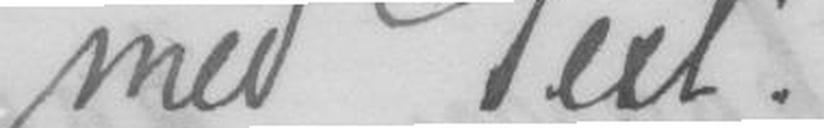med Text?
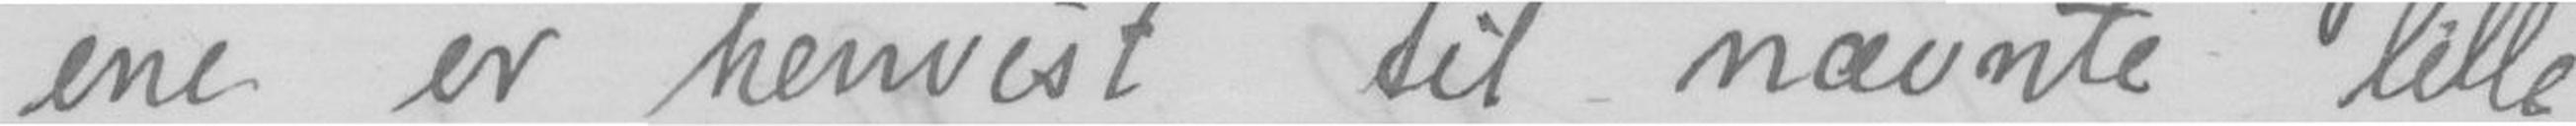ene er henvist til nævnte lille
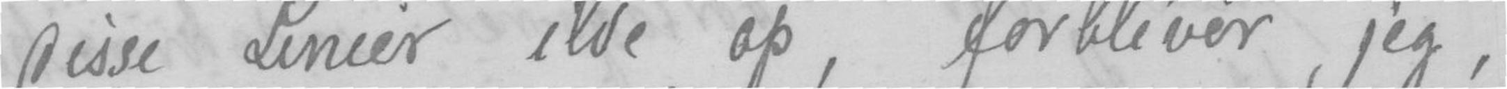Disse Linier ilde op, forbliver jeg,
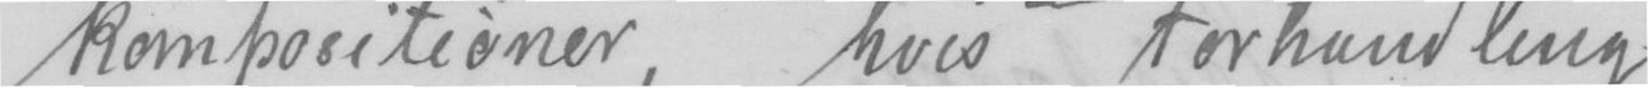kompositioner, hvis Forhandling-
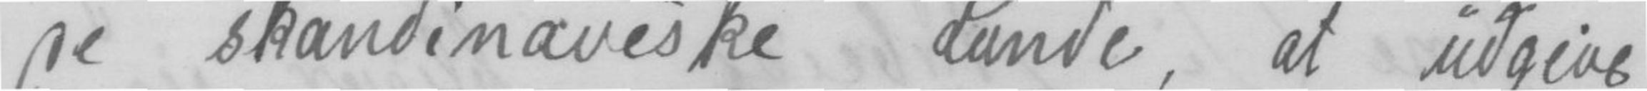De skandinaviske Lande, at udgive
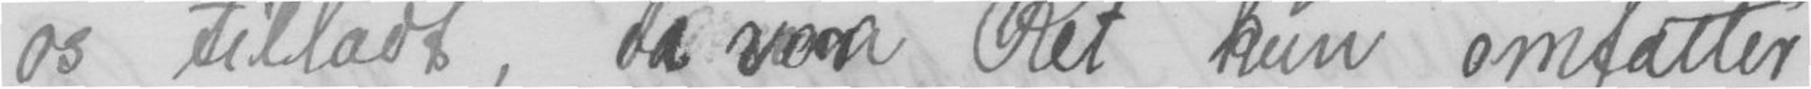os tilladt, da Ret kun omfatter
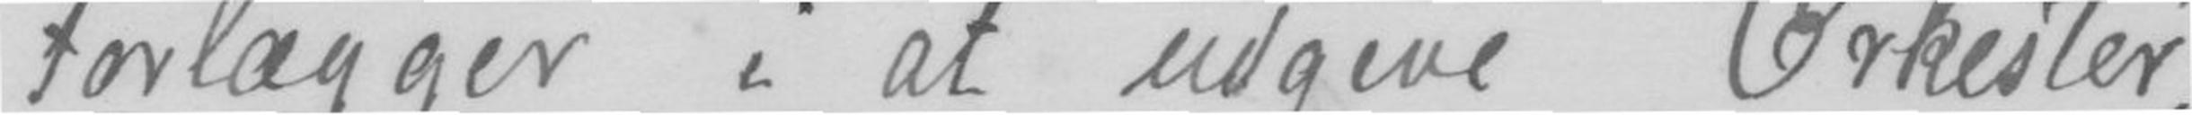Forlægger i at udgive Orkester-
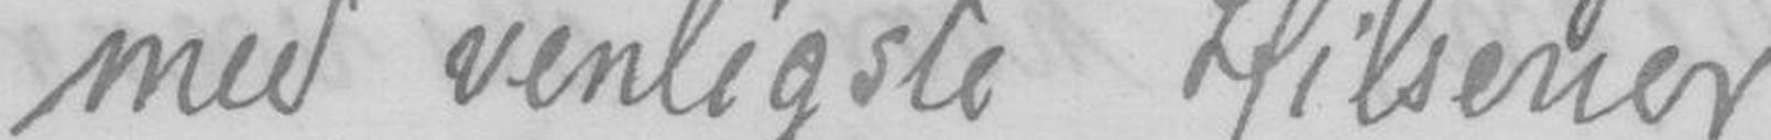med venligste Hilsener
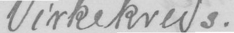Virkekreds.
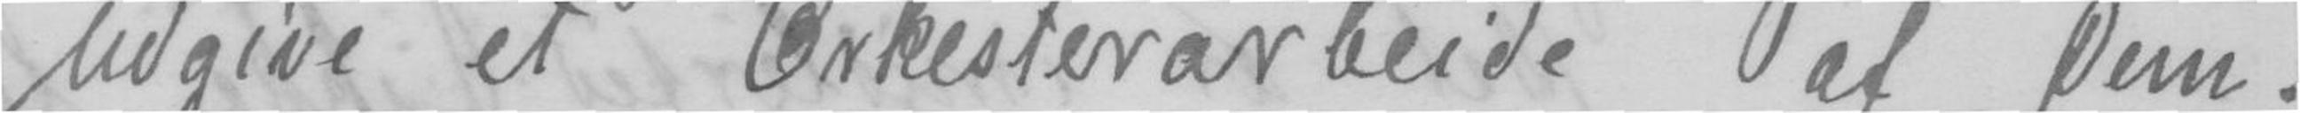udgive et Orkesterarbeide af Dem.
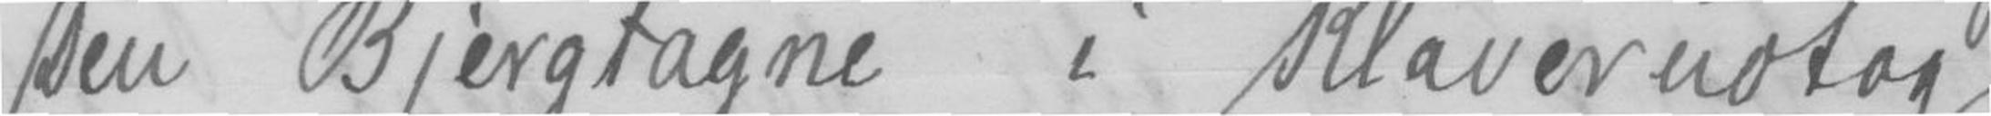Den Bjergtagne i Klaverudtag
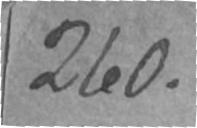260.
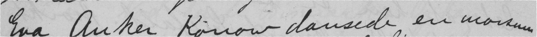Eva Anker Konow dansede en morsom
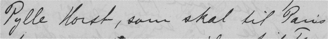Pylle Horst, som skal til Pans
